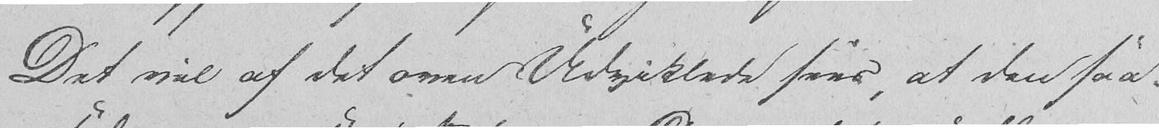Det vil af det oven Udviklede sies, at den saa-
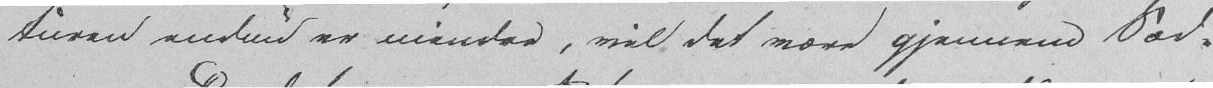turen endnu er mindre, vil det være gjennem Sæd-
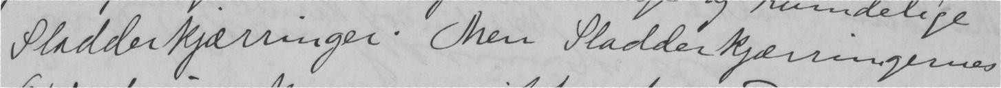Sladderkjærringer. Men Sladderkjærringernes
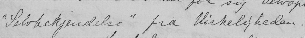"Selvbekjendelse" fra Virkeligheden.
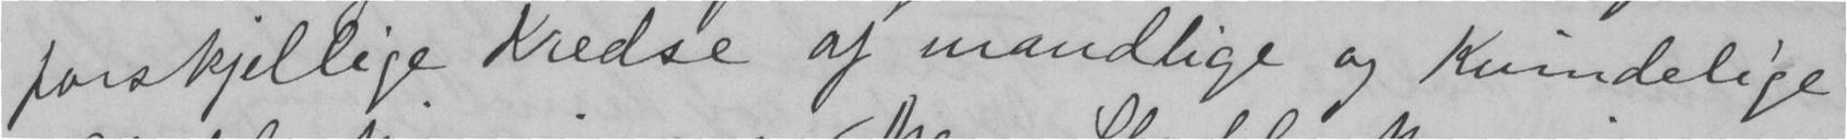forskjellige Kredse af mandlige og Kvindelige
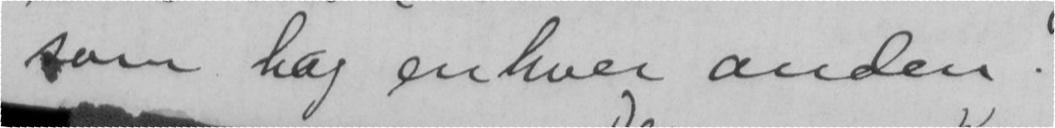som bag enhver anden.
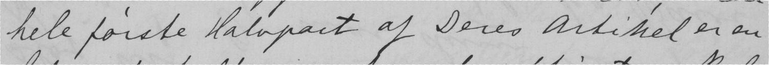hele første Halvpart af Deres Artikel er en
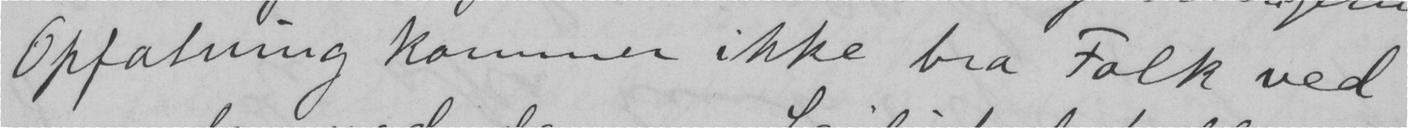Opfatning kommer ikke bra Folk ved
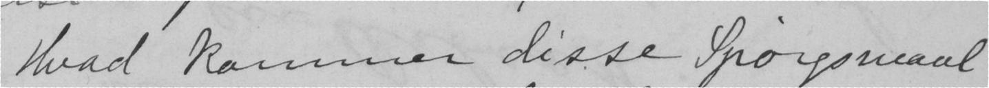Hvad kommer disse Spørgsmaal
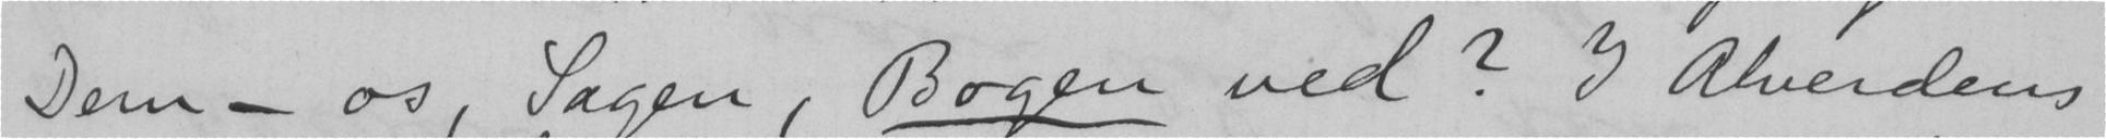Dem - os, Sagen, Bogen ved? I Alverdens
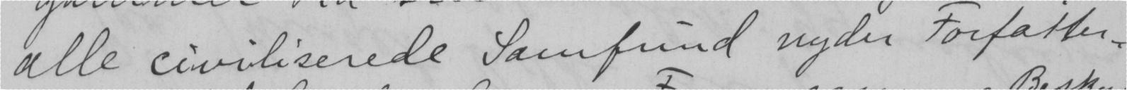alle civiliserede Samfund nyder Forfatter-
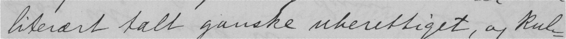literært talt ganske uberettiget, og kul-
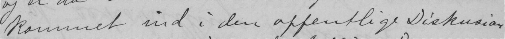kommet ind i den offentlige Diskusion
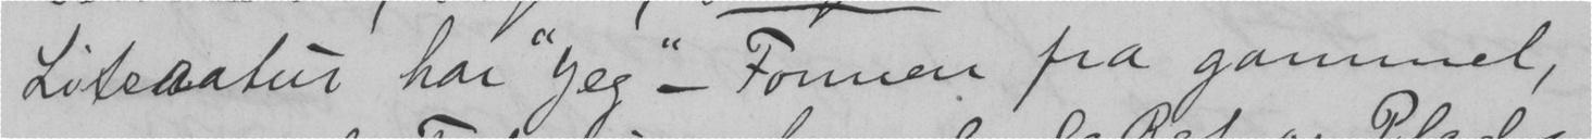Literatur har "jeg"-Formen fra gammel,
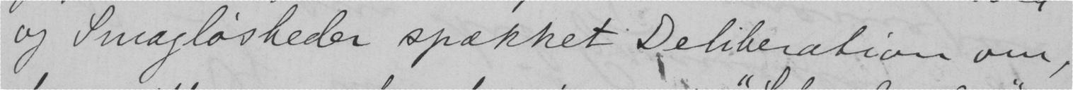og Smagløsheder spækket Deliberation om,
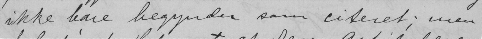ikke bare begynder som citeret; men
